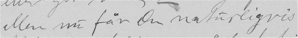Men nu får Du naturligvis
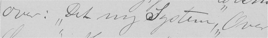over: "Det ny System", "Over
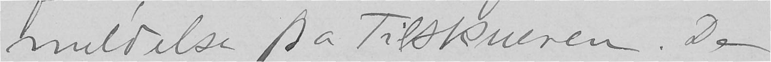meldelse på Tilskueren. De
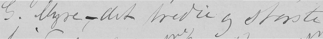G. Myre - det tredie og største
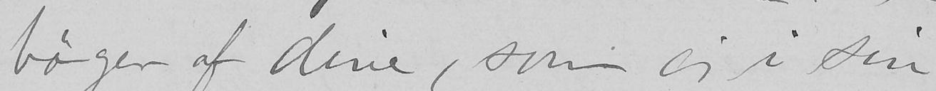bøger af dine, som jeg i sin
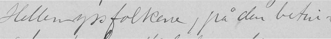Hellemyrsfolkene, på den betin-
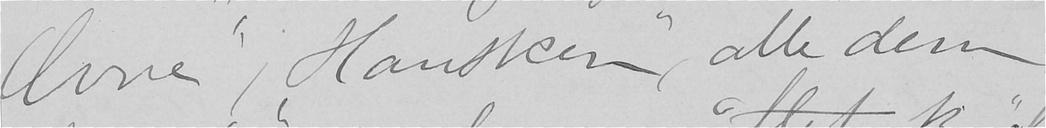Ævne", "Hansken", alle dem
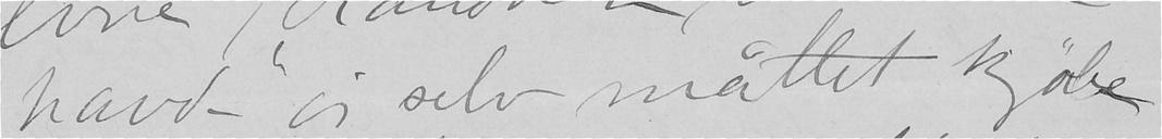havde jeg selv måttet kjøbe
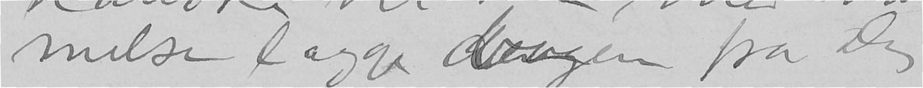melse lægge bogen fra Dig.
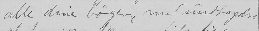alle dine bøger, med undtagelse
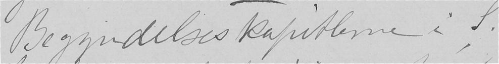Begyndelses kapitterne i S.
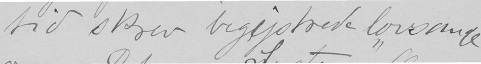tid skrev begejstrede lovsange
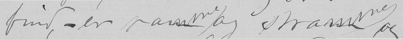bind - er vamme og stramme og
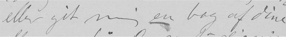eller git mig en bog af dine
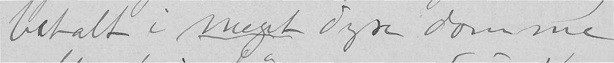betalt i meget dyre domme
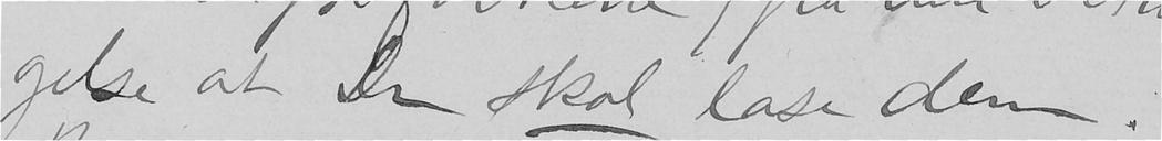gelse at Du skal læse dem.
